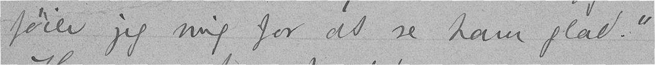føier jeg mig for at se ham glad."
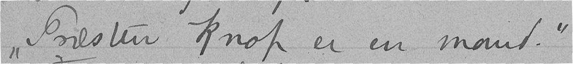"Præsten Knop er en mand."
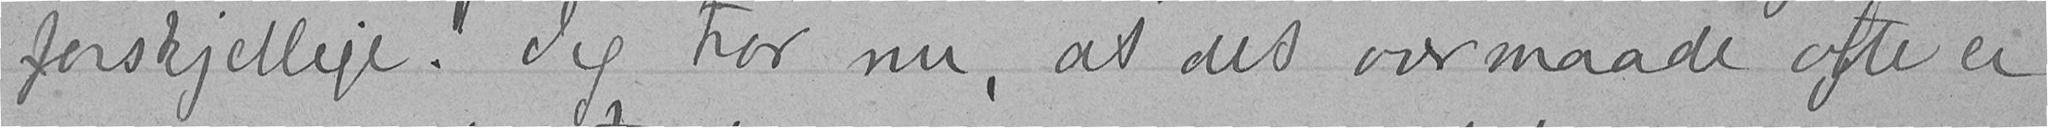forskjellige. Jeg tror nu, at det overmaade ofte er
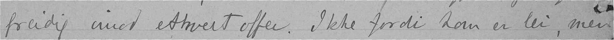freidig imod ethvert offer. Ikke fordi han er lei, men
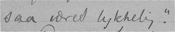saa været lykkelig."
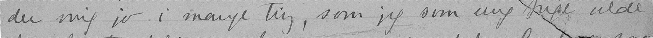der mig jo i mange ting, som jeg som ung pige vilde
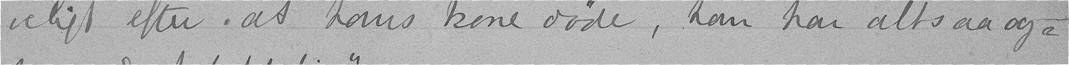veligt efter at hans kone døde, han har altsaa og-
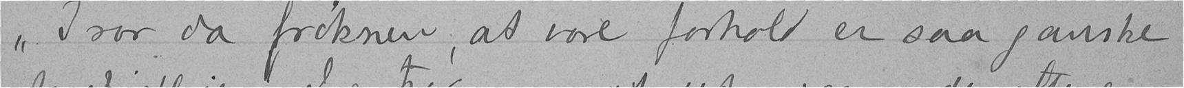"Tror da frøknen, at vore forhold er saa ganske
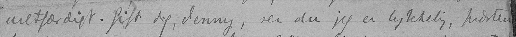uretfærdigt. Gift dig, Jenny, ser du jeg er lykkelig, præsten
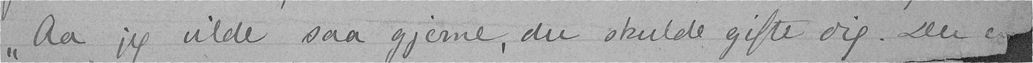"Aa jeg vilde saa gjerne, du skulde gifte dig. Der er
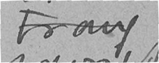trang
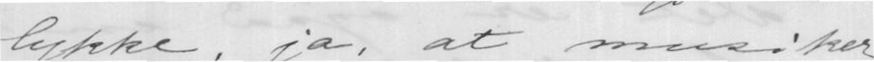lykke, ja, at musiker-
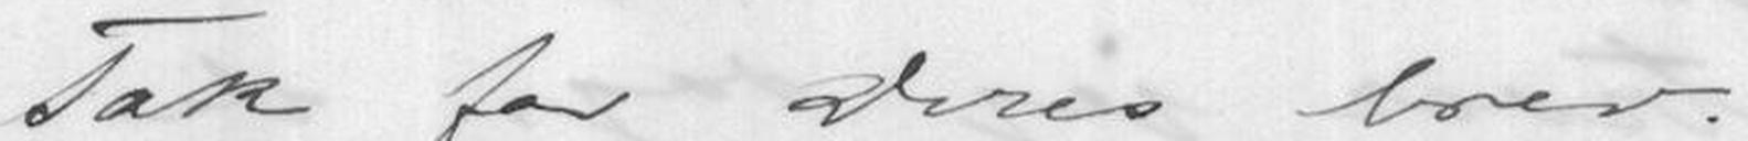Tak for Deres brev.
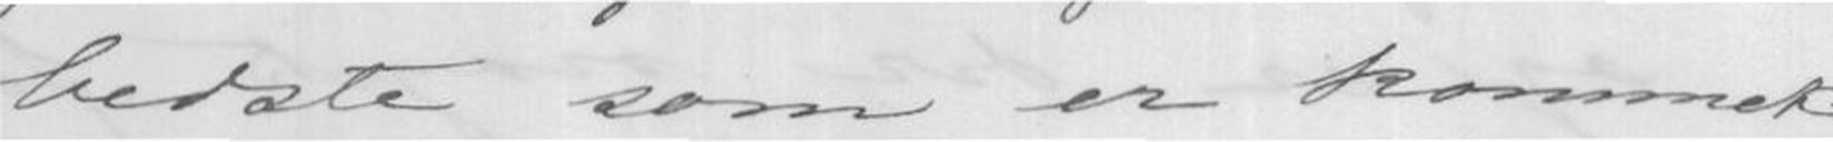bedste som er kommet
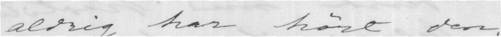aldrig har hørt den,
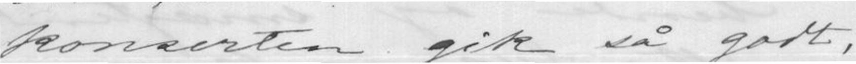konserten gik så godt,
